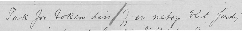Tak for boken din. Jeg er netop blit færdig
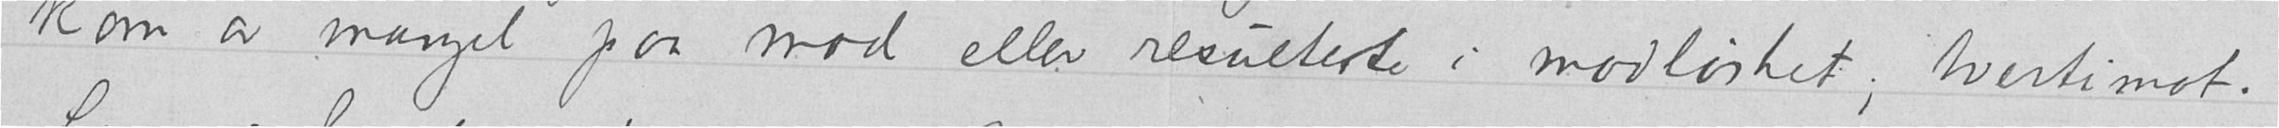kom av mangel paa mod eller resulterte i modløshet; tvertimot.
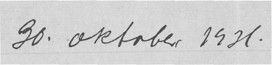30. oktober 1931.
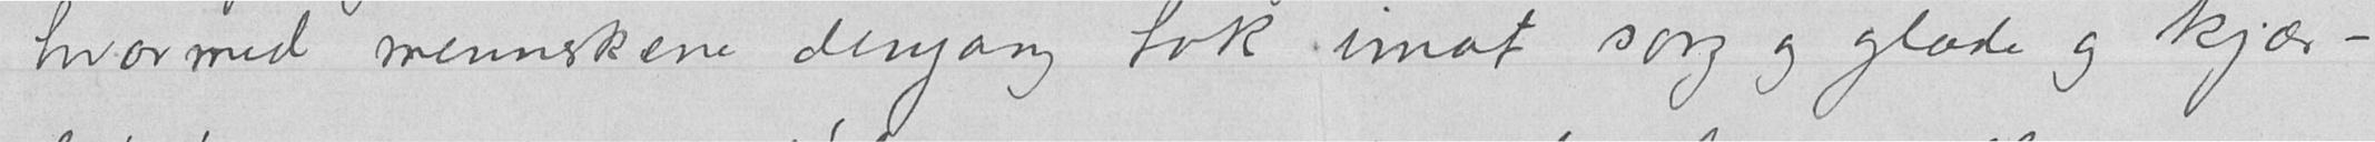hvormed menneskene dengang tok imot sorg og glæde og kjær-
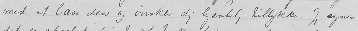med at læse den og ønsker dig hjertelig tillykke. Jeg synes
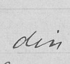din
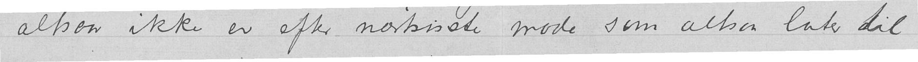altsaa ikke er efter næstsisste mode som altsaa later til
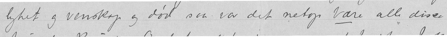lighet og venskap og død saa var det netop bare alle disse
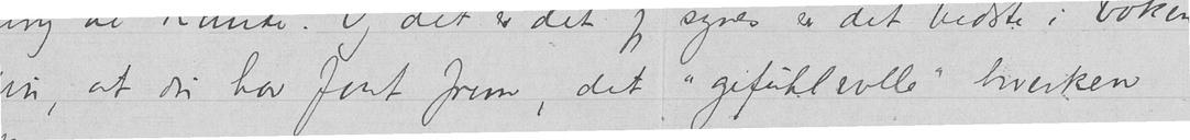din, at du har faat frem, det "gefühlwolle" hverken
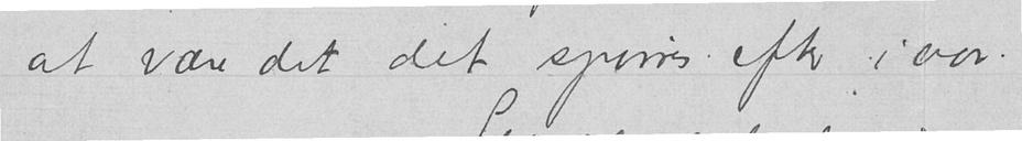at være det det spørres efter i aar.
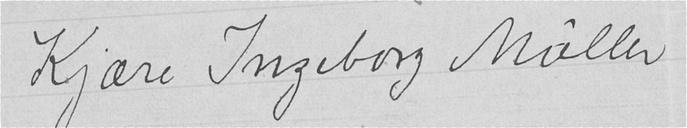Kjære Ingeborg Møller
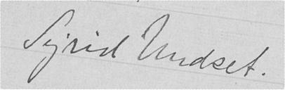Sigrid Undset.
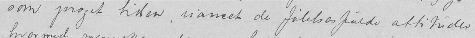som præget tiden, uancet de følelsesfulde attituder
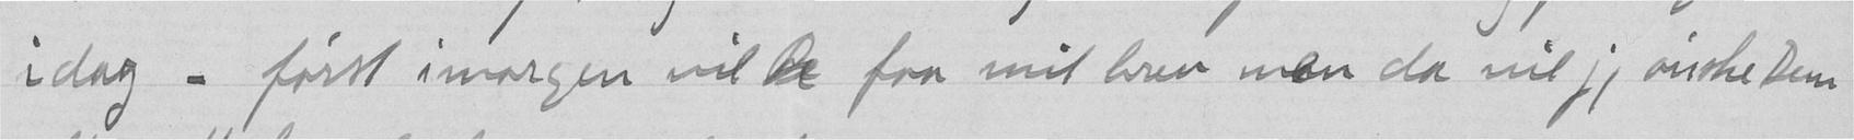idag - først imorgen vil De faa mit brev men da vil jeg ønske Dem
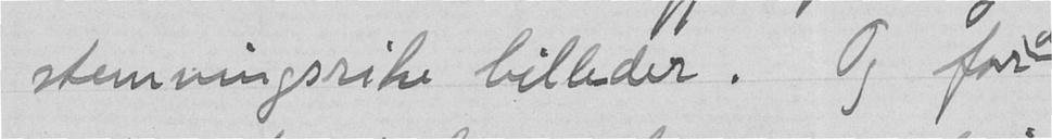stemningsrike billeder. Og for
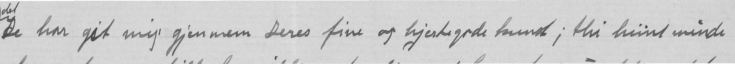De har git mig gjennem Deres fine og hjertegode kunst; thi hiint minde
